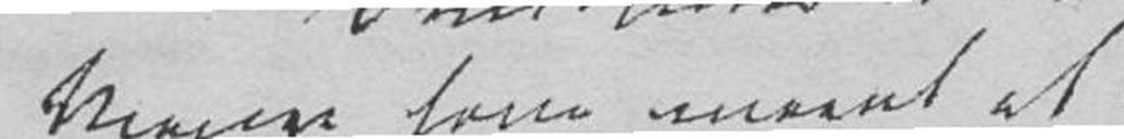Mange have meent at
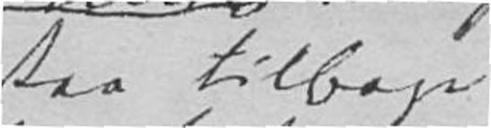staa tilbage
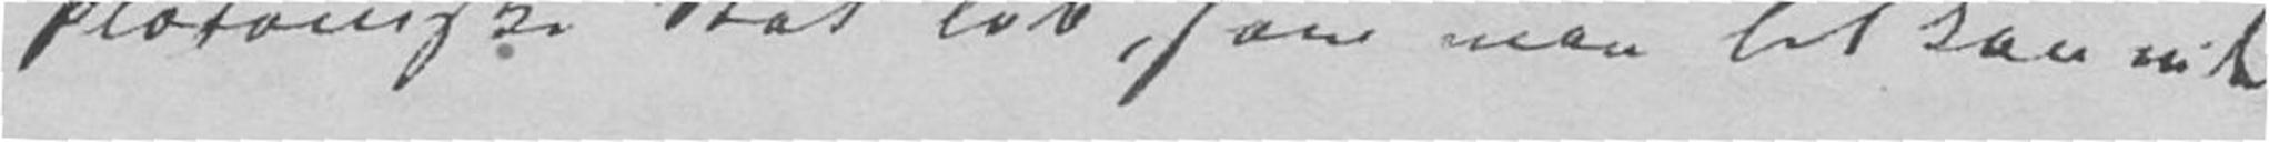platoniske Stat løb, som man let kan vide
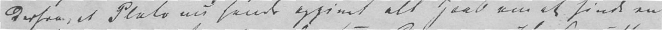derfra, at Plato nu havde opgivet alt Haab om at finde en
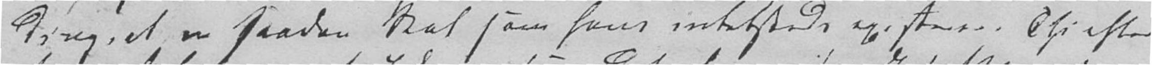ding, at en saadan Stat som hans intetsteds existere. Thi efter
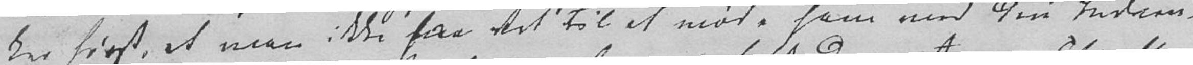ker først, at man ikke faa Ret til at møde ham med den Indven-
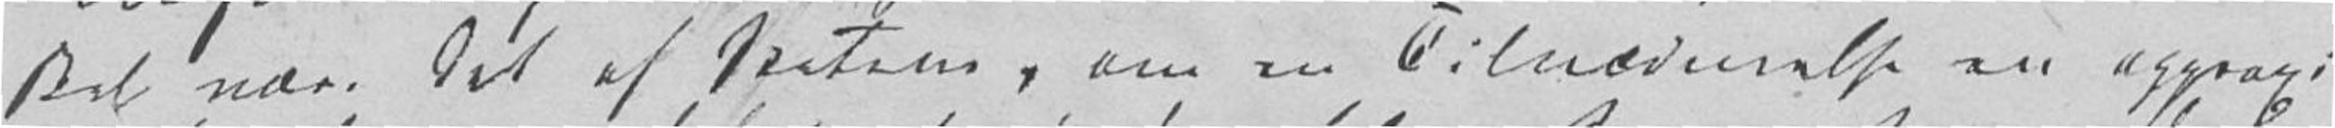skal være det af Statens, om en Tilnærmelse en approxi-
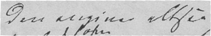den angiver altsaa
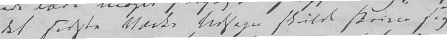det sidste Værks Udsagn skulde skrive sig
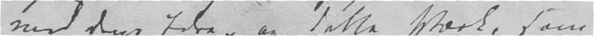med dens Idee, og dette Værk, som
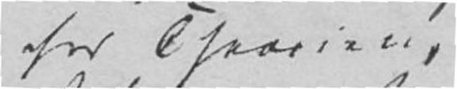for Theorien,
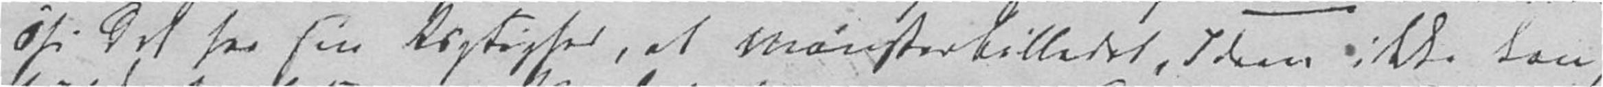Thi det har sin Rigtighed, at Mønsterbilledet, Ideen ikke kan
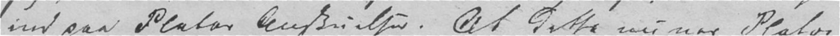ind paa Platos Anskuelser. At dette nu var Platos
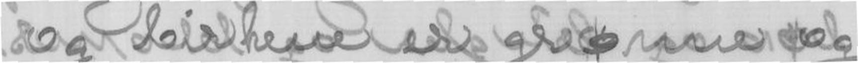og birkene er grønne og
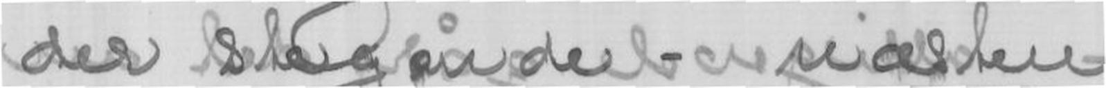der stegende - næsten
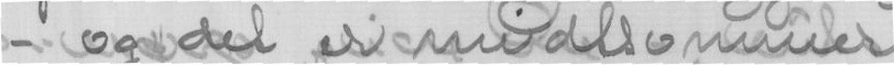- og det er midtsommer
